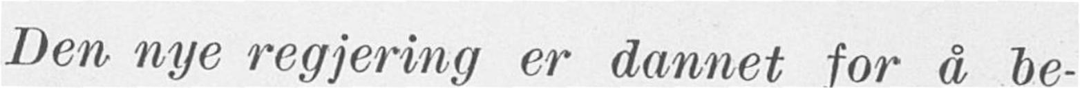Den nye regjering er dannet for å be-
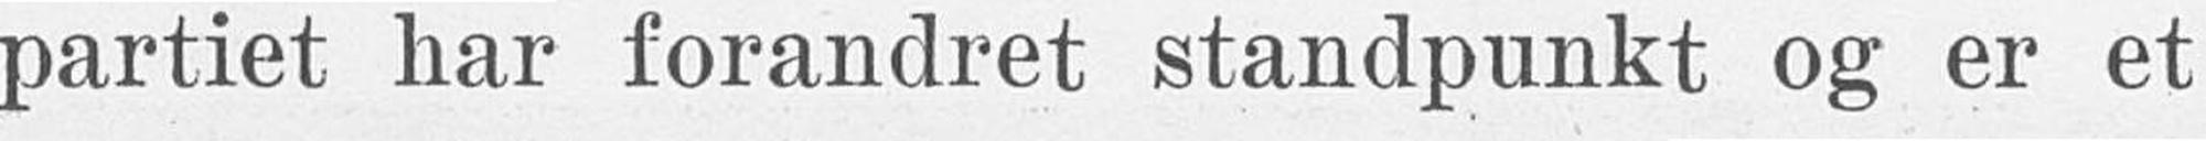partiet har forandret standpunkt og er et
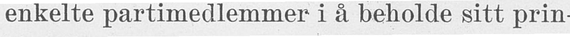enkelte partimedlemmer i å beholde sitt prin-
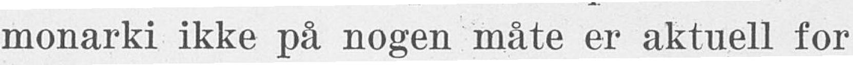monarki ikke på nogen måte er aktuell for
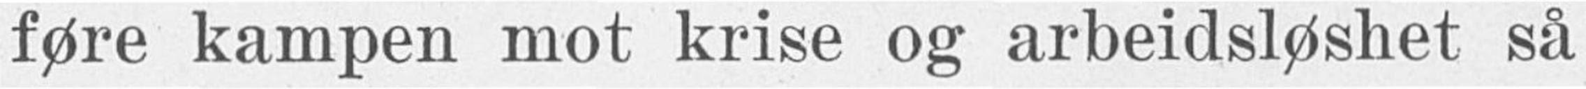føre kampen mot krise og arbeidsløshet så
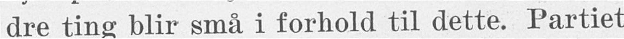dre ting blir små i forhold til dette. Partiet
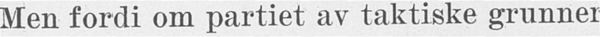Men fordi om partiet av taktiske grunner
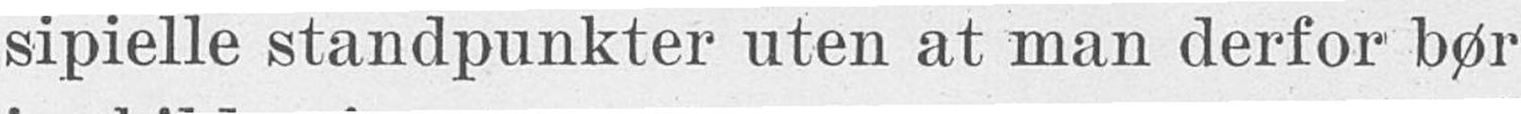sipielle standpunkter uten at man derfor bør
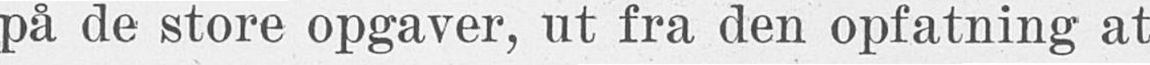pâ de store opgaver, ut fra den opfatning at
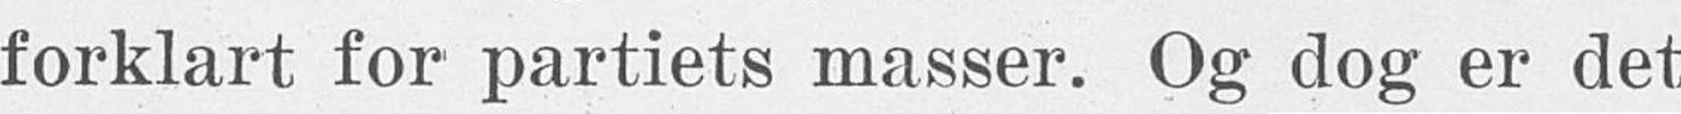forklart for partiets masser. Og dog er det
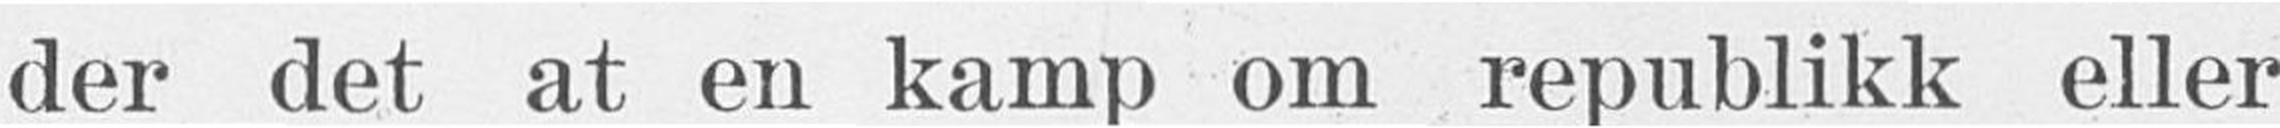der det at en kamp om republikk eller
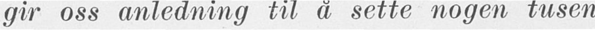gir oss anledning til å sette nogen tusen
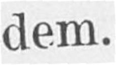dem.
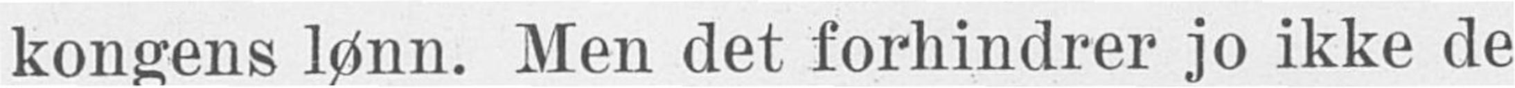kongens lønn. Men det forhindrer jo ikke de
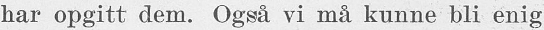har opgitt dem. Også vi må kunne bli enig
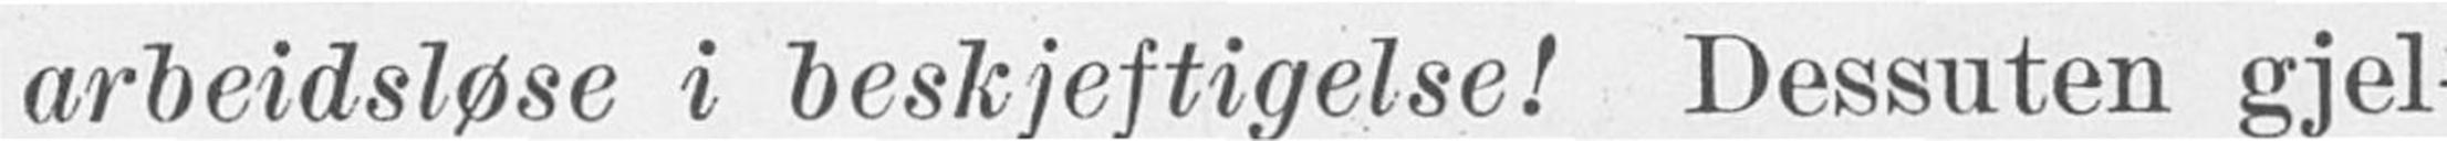arbeidsløse i beskjeftigelse! Dessuten gjel-
